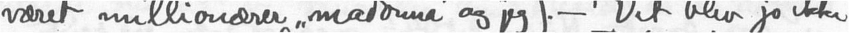været millionærer "Madonna" og jeg). - Det blev jo ikke
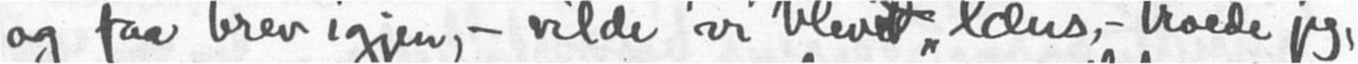og faa brev igjen, - vilde vi blevet "læns",- troede jeg,
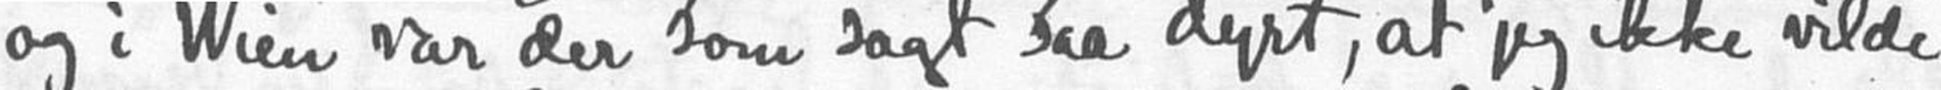og i Wien var der som sagt saa dyrt, at jeg ikke vilde
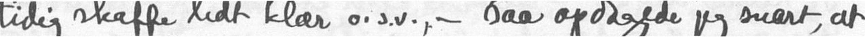tidig skaffe lidt klær o.s.v., - saa opdagede jeg snart, at
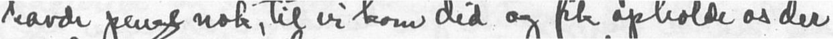havde penge nok, til vi kom did og fik opholde os der
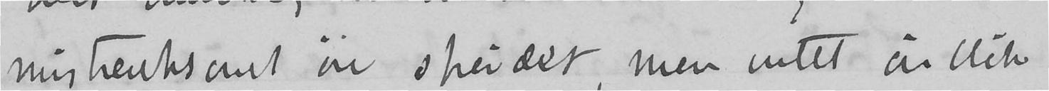mistenksomt øie speidet, men intet øieblik
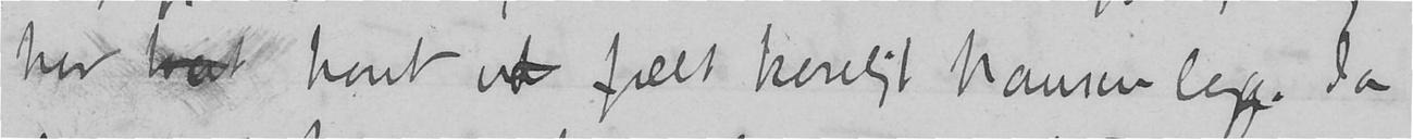har hat havt et fælt koseligt Nansen lag. Ja
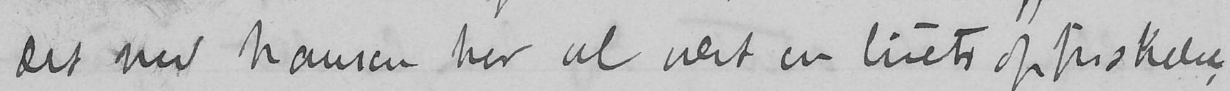det med Nansen har vel vært en livets opfriskelse,
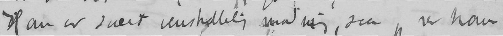Han er svært venskabelig med mig, saa jeg ser ham
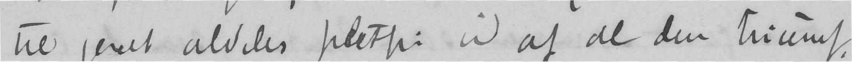til gaat aldeles pletfri ud af al den triumf,
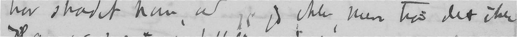har skadet ham, ved jeg jo ikke, men tror det ikke
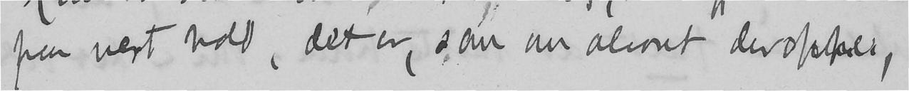paa nært hold, det er, som om alvoret deroppe,
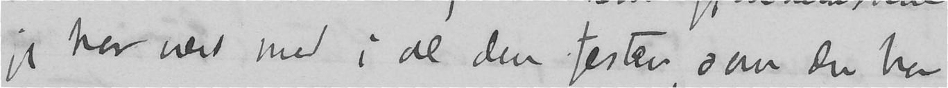jeg har vært med i al den festen som der har
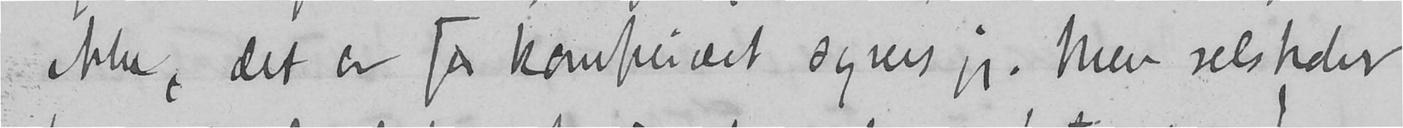ikke, det er for kompliceret synes jeg. Men selskaber
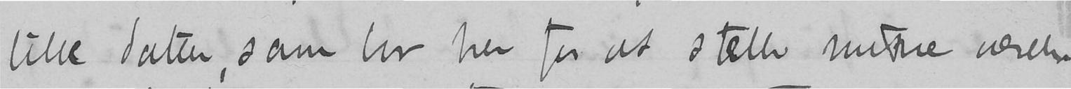lille datter, som bor her for at stælle mine værelser
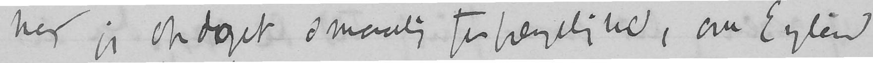har jeg opdaget smaalig forfængelighed, om England
